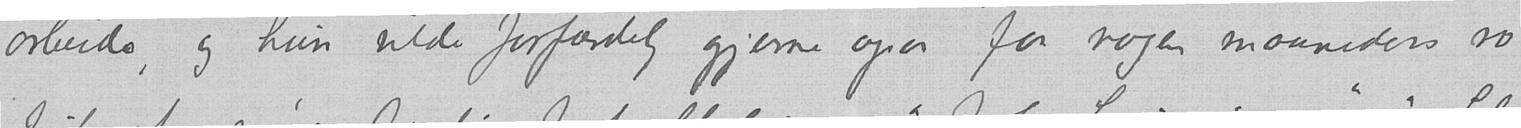arbeide og hun vilde forfærdelig gjerne ogsaa faa nogen maaneders ro
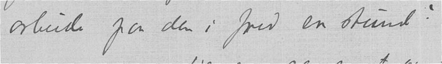arbeide paa den i fred en stund?
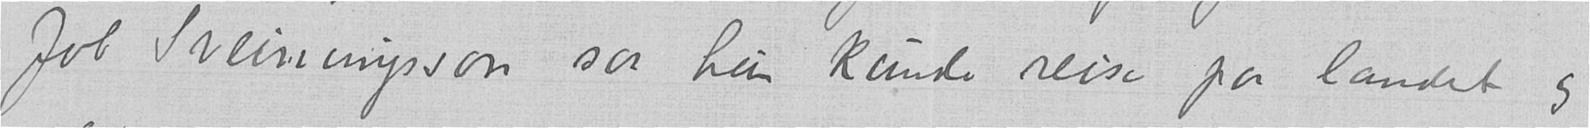Job Sveiningsson saa hun kunde reise paa landet og
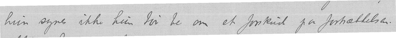hun synes ikke hun tør be om et forskud paa fortsættelsen.
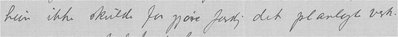hun ikke skulde faa gjøre færdig det planlagte verk.
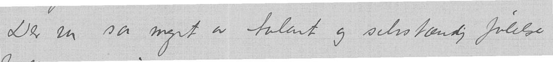Der var saa meget av talent og selvstændig følelse
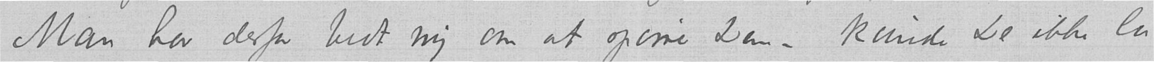Man har derfor bedt mig om at spørre Dem – kunde De ikke la
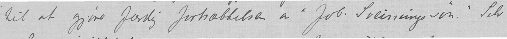til at gjøre færdig fortsættelsen av "Job Sveinungsson." Selv
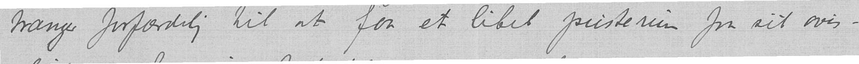trænger forfærdelig til at faa et litet pusterum fra sit avis-
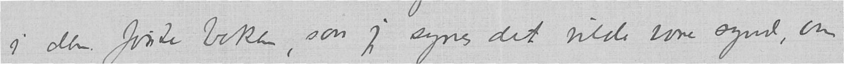i den første boken, saa jeg syns det vilde være synd, om
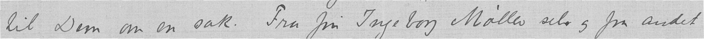til Dem om en sak. Fra fru Ingeborg Møller selv og fra andet
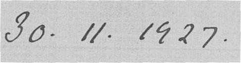30.11.1927.
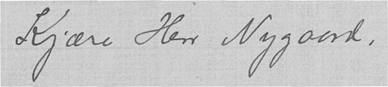Kjære Herr Nygaard,
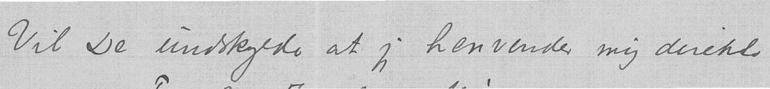Vil De undskylde at jeg henvender mig direkte
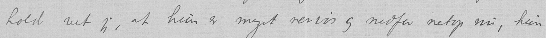hold vet jeg, at hun er meget nervøs og nedfor netop nu, hun
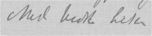Med bedste hilsen
